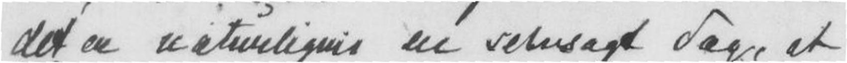det er naturligvis en selvsagt Sag, at
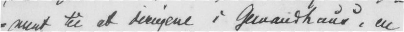ment til at dirigere i Grandhaus, ere
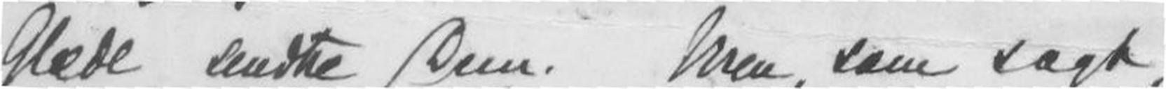Glæde sendte Dem. Men, som sagt
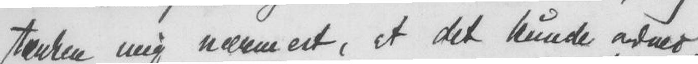tænker mig nærmest, at det kunde ,
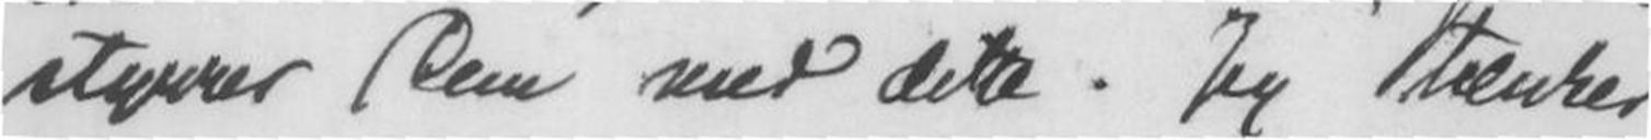stykker Dem med dette. Jeg tænker
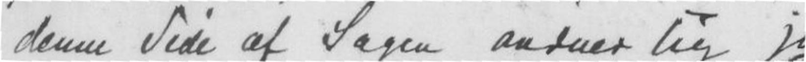denne Side af Sagen lig jo
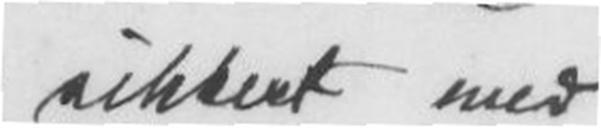sikkert med
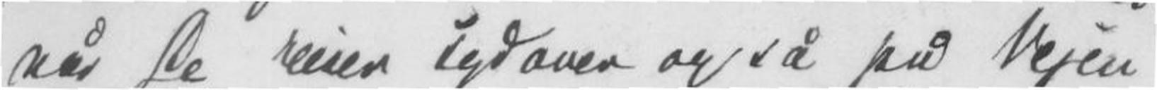når De reiser Sydover og så på Vejen
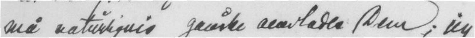må naturligvis Dem; jeg
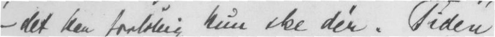- det kan foreløbig kun ske der. Tiden
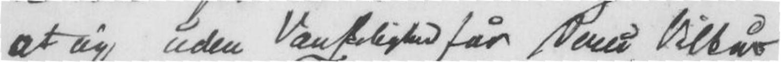at jeg uden Vanskelighed får tilbud
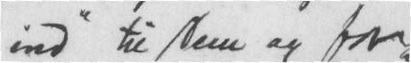ind" til Dem og for-
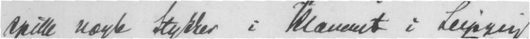spille nogle stykker i Klaveret i Leipzig,
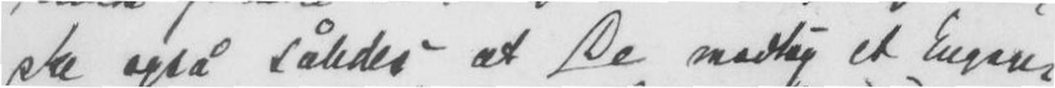ske også således, at De nodtog et Engage-
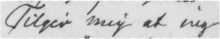Tilgiv mig at jeg
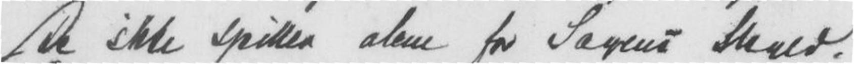De ikke spiller alene for Sagens skyld.
